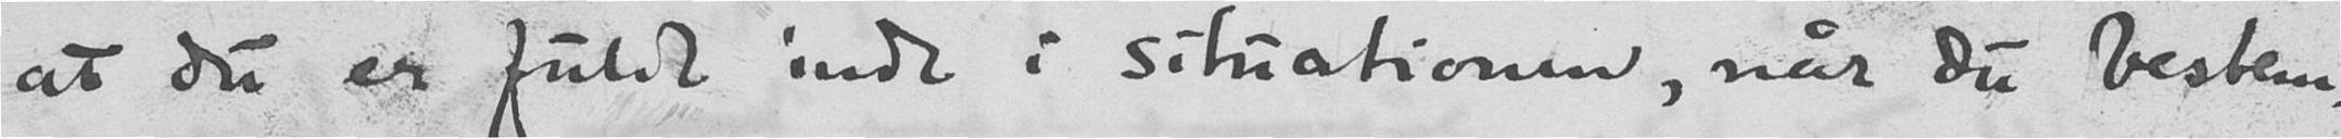at du er fuldt inde i situationen, når du bestem-
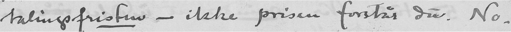talingsfristen - ikke prisen forstår du. No-
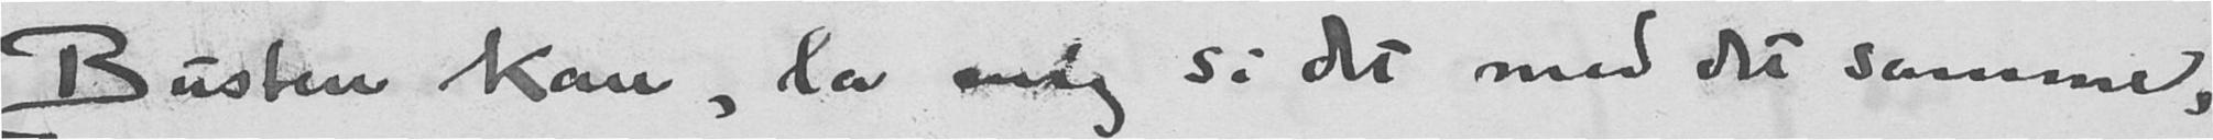Busten kan, la mig si det med det samme,
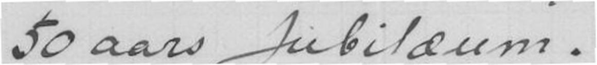50 aars Jubilæum.
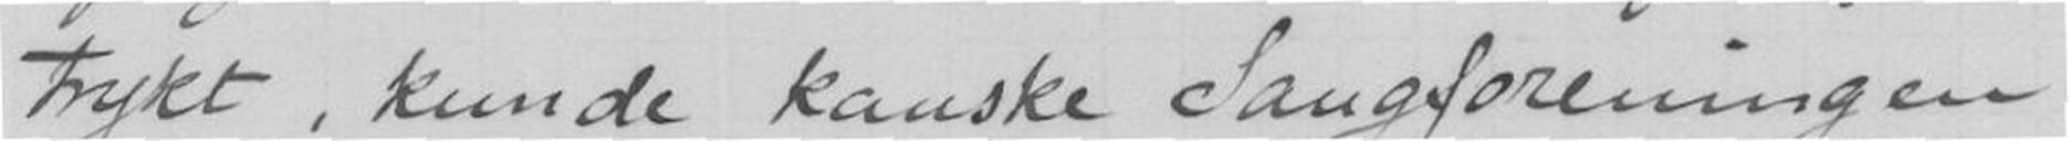trykt, kunde kanske Sangforeningen
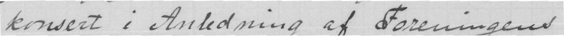konsert i Anledning af Foreningens
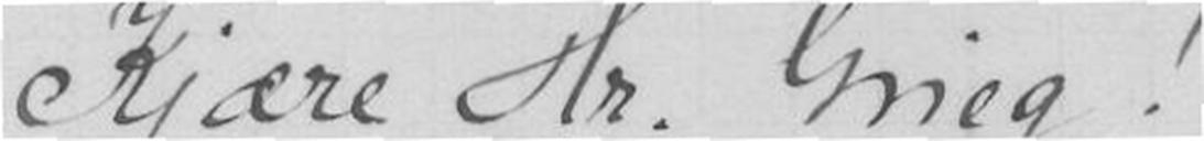Kjære Hr. Grieg!
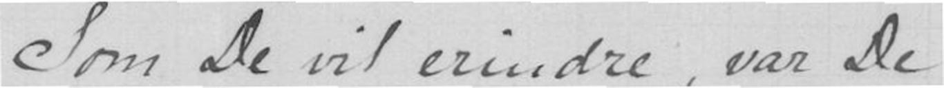Som De vil erindre, var De
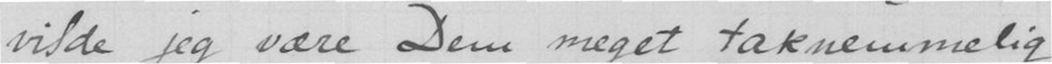vilde jeg være Dem meget taknemmelig,
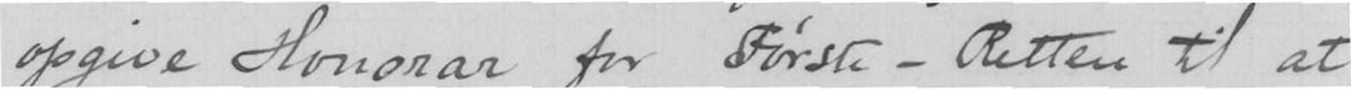opgive Honorar for Første - Retten til at
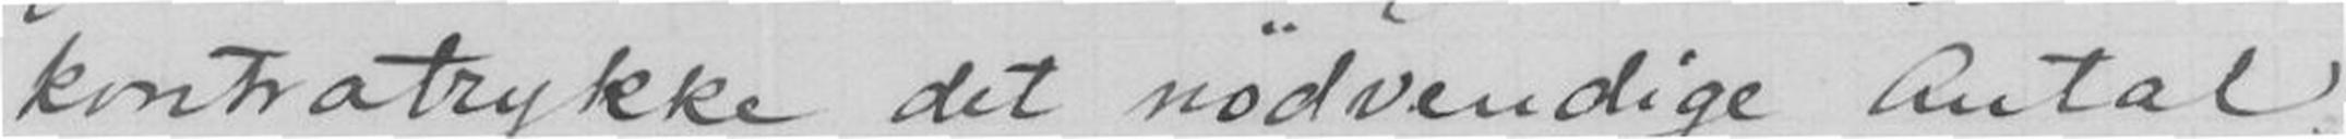kontrotrykke det nødvendige Antal
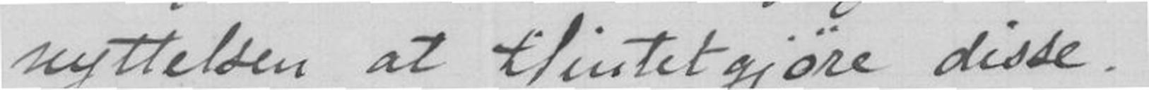nyttelsen at tilintetgjøre disse.
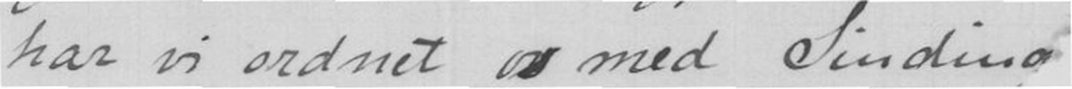har vi ordnet med Sinding
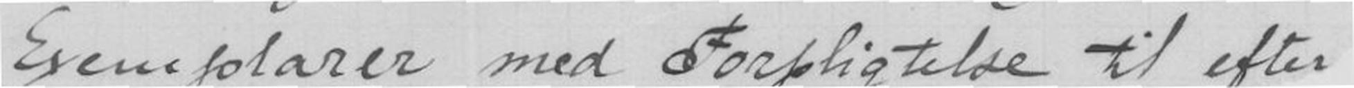Exemplarer med Forjtigtelse til efter afbe-
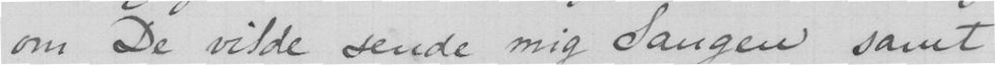om De vilde sende mig Sangen samt
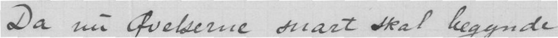Da nu Øvelsene snart skal begynde,
